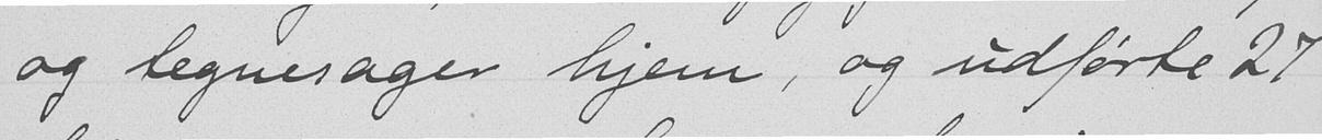og tegnesager hjem, og udførte 27
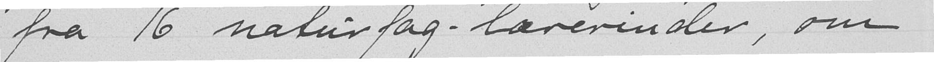fra 16 naturfag-lærerinder, om
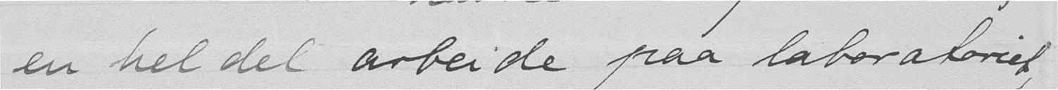en hel del arbeide paa laboratoriet,
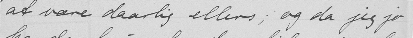at være daarlig ellers; og da jeg jo
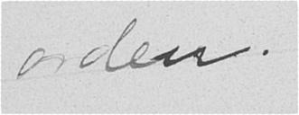orden.
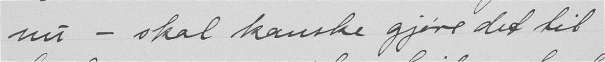nu - skal kanske gjøre det til
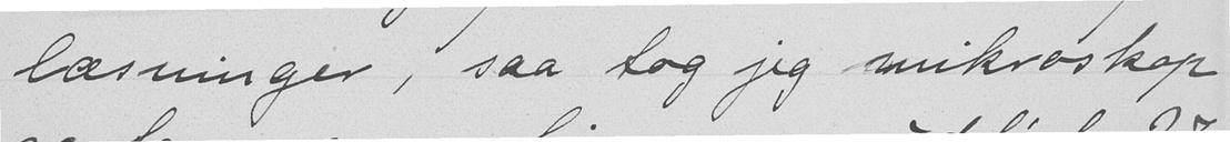læsninger, saa tog jeg mikroskop
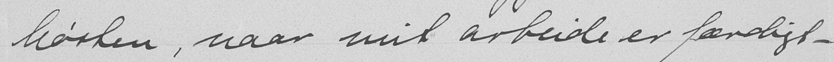høsten, naar mit arbeide er færdigt -
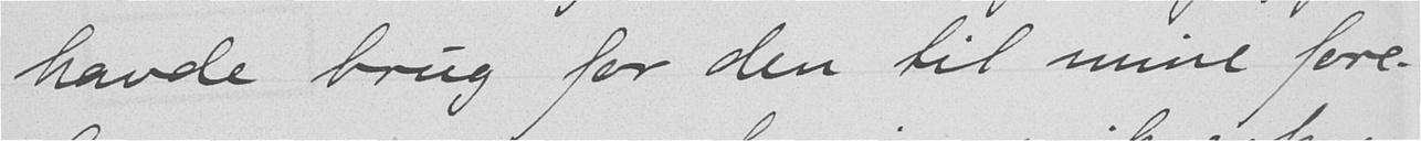havde brug for den til mine fore-
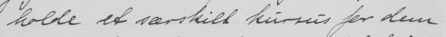holde et særskilt kursus for dem
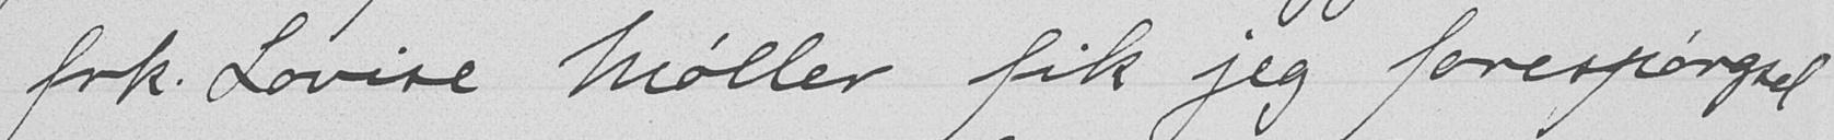frk. Lovise Møller fik jeg forespørgsel
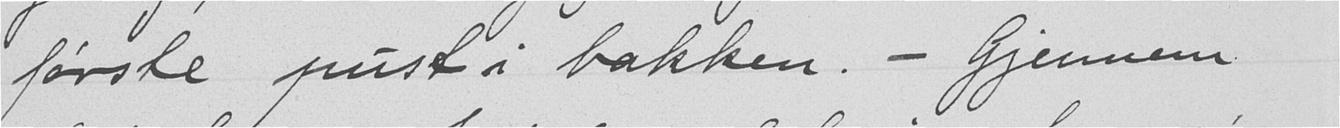første pust i bakken. - Gjennem
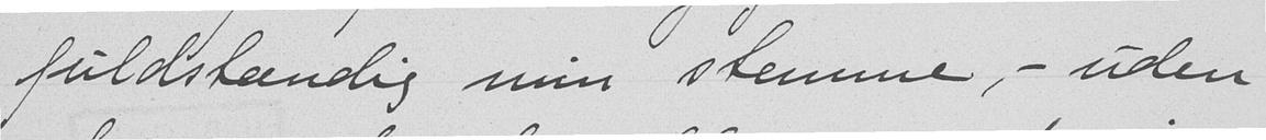fuldstændig min stemme, - uden
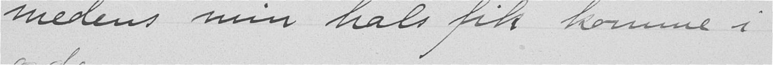medens min hals fik komme i
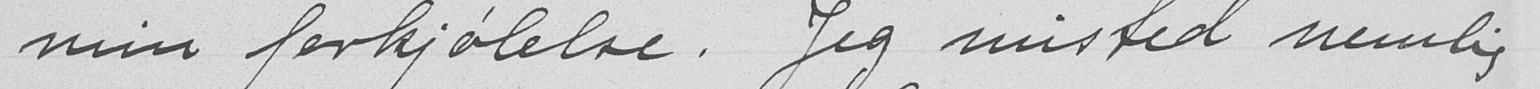min forkjølelse. Jeg misted nemlig
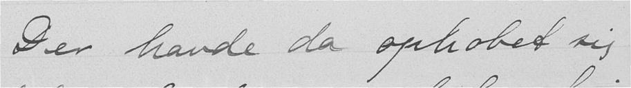Der havde da ophobet sig
